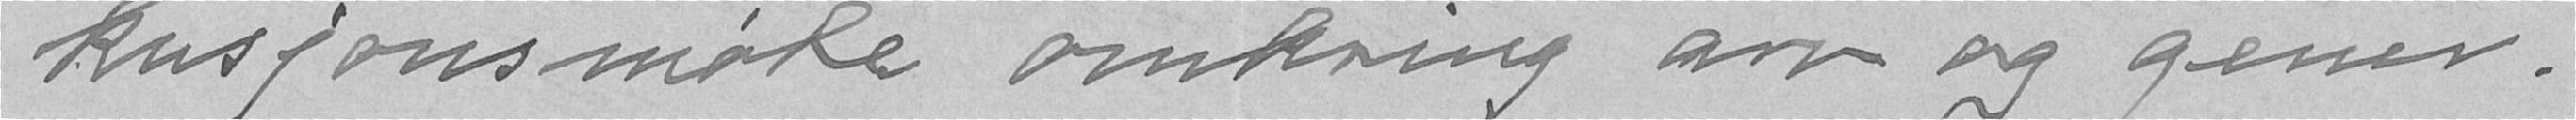kusjonsmøte omkring arv og gener.
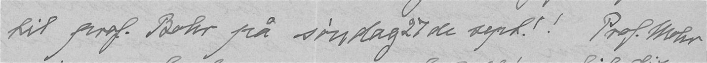til prof. Bohr på søndag 27de sept.! Prof. Mohr
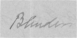Blindern
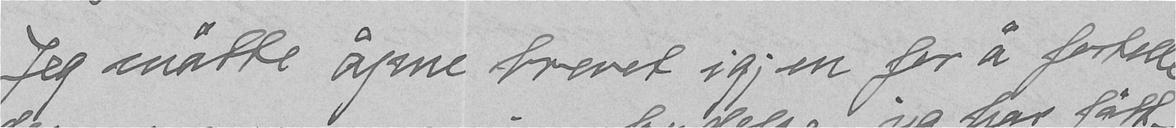Jeg måtte åpne brevet igjen for å fortelle
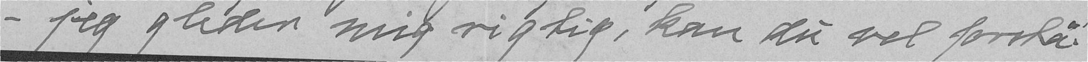- jeg gleder mig rigtig, kan du vel forstå!
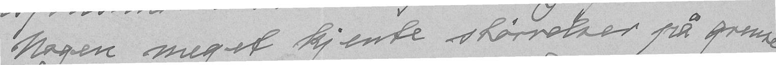Nogen meget kjente størrelser på grense-
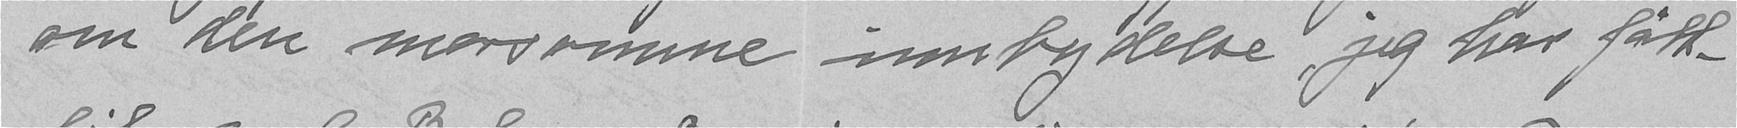om den morsomme innbydelse, jeg har fått -
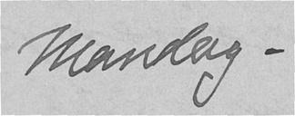Mandag -
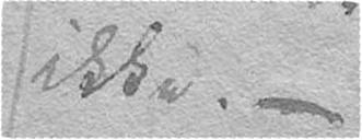ikke. –
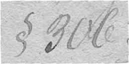§ 306.
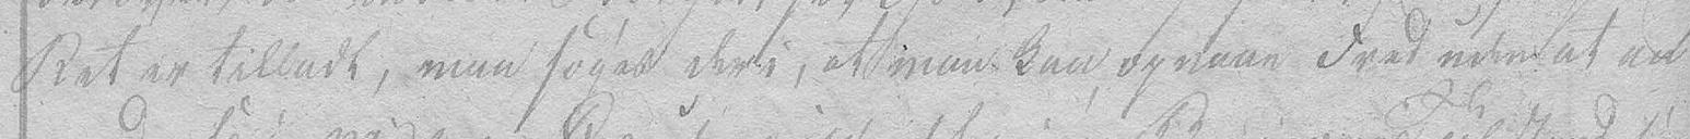Ret er tilladt; maa søges deri, at man kan opnaae Fred uden at an-
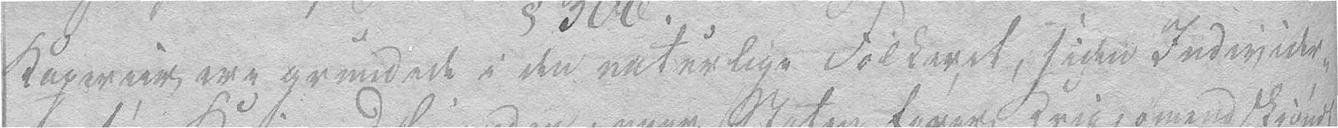Kaperier ere grundede i den naturlige Folkeret, siden Individer-
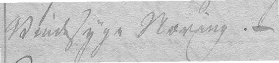Vindesyge Næring. –
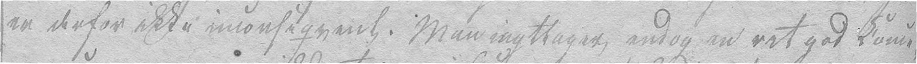er derfor ikke inconseqvent. Man iagttager endog en ret god Conce-
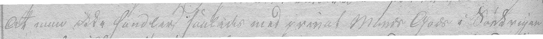At man ikke handler saaledes med privat Mands Gods i Søekrigen
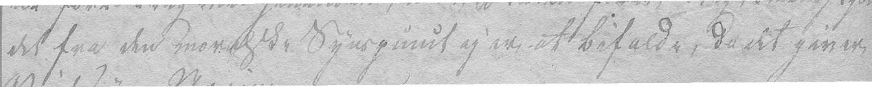det fra den moralske Synspunct ey er at bifalde, da det giver
